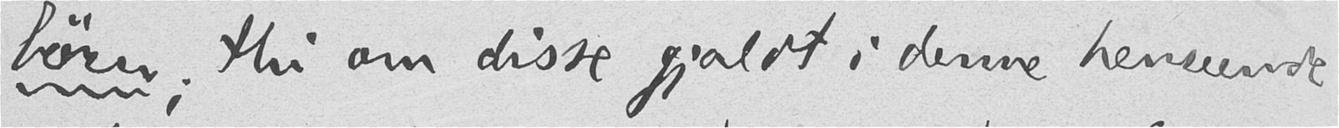børn; thi om disse gjaldt i denne henseende
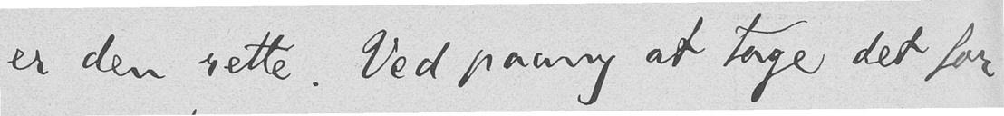er den rette. Ved paany at tage det for
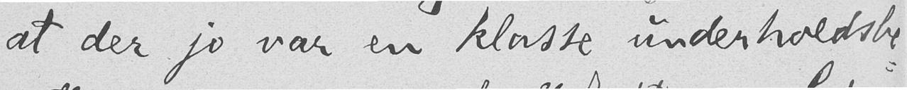at der jo var en klasse underholdsbe-
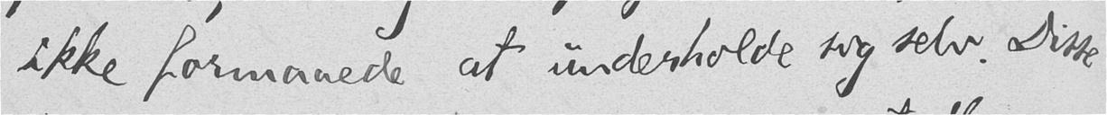ikke formaaede at underholde sig selv. Disse
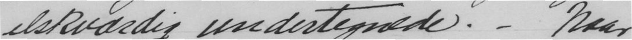elskværdig undertegnede. - Naar
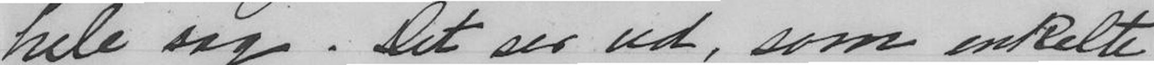hele sag. Det ser ud, som enkelte
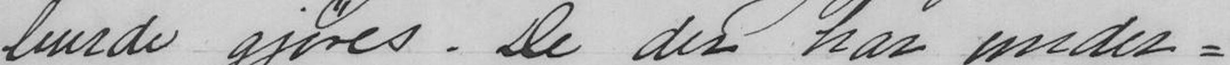burde gjøres. De der har under-
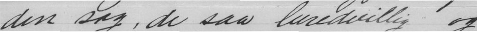den sag, de saa beredvillig og
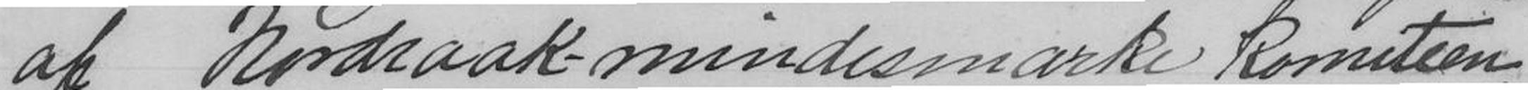af Nordraak-mindesmærke komiteen.
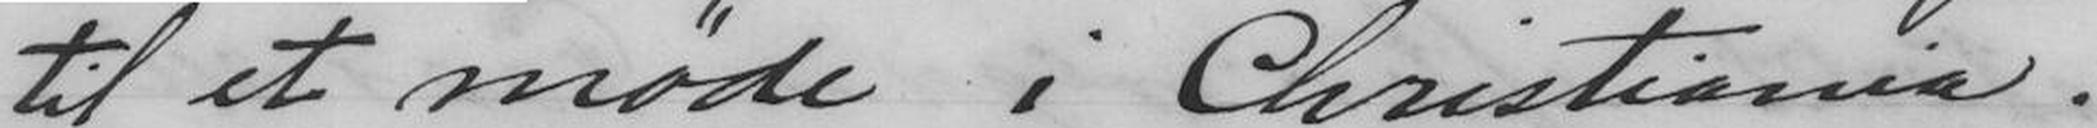til et møde i Christiania.
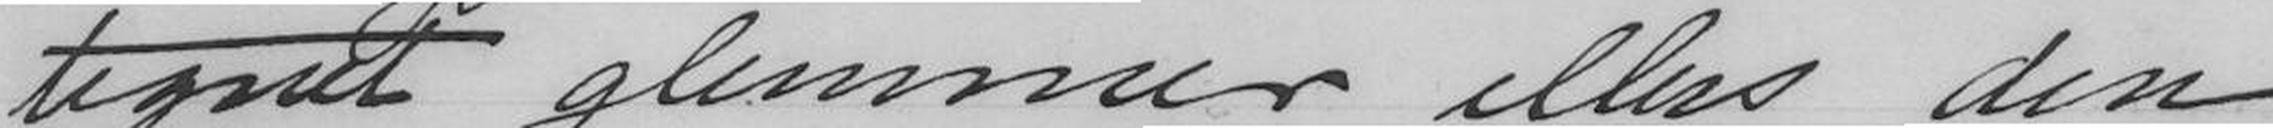tegnet glemmer ellers den
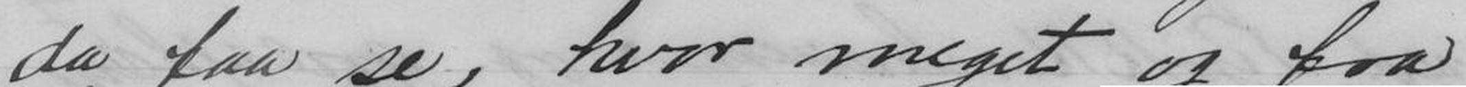da faa se, hvor meget og fra
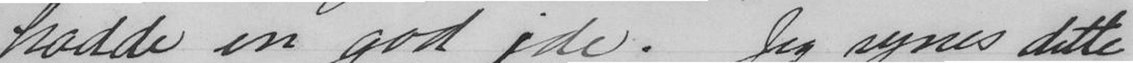hadde en god ide. Jeg synes dette
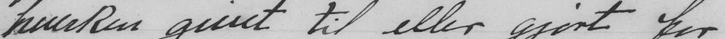hverken givet til eller gjort for
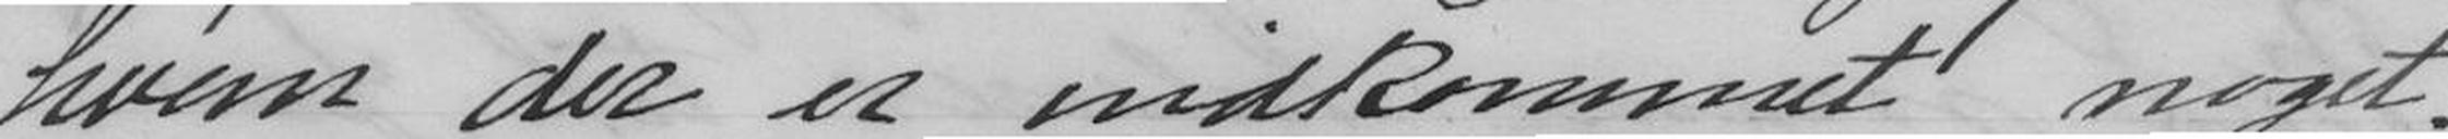hvem der er indkommet noget.
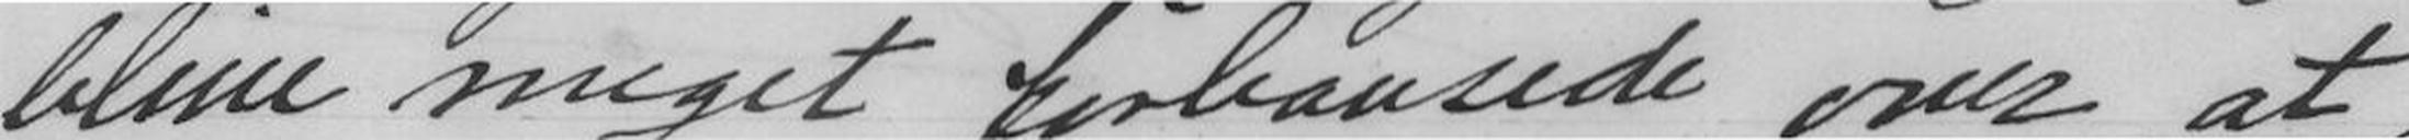blive meget forbausede over at
